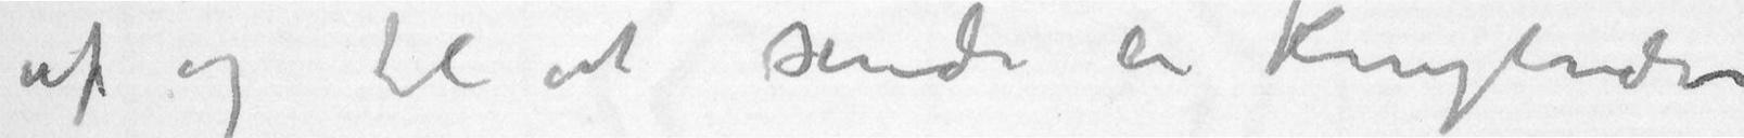af og til at sende en Knytnæve
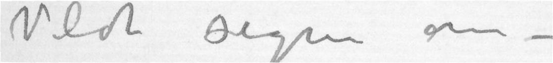Vildt segne om –
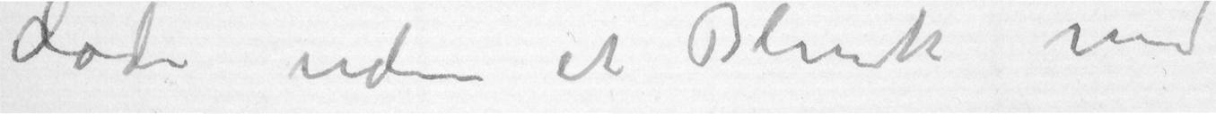døde uden et Blunk med
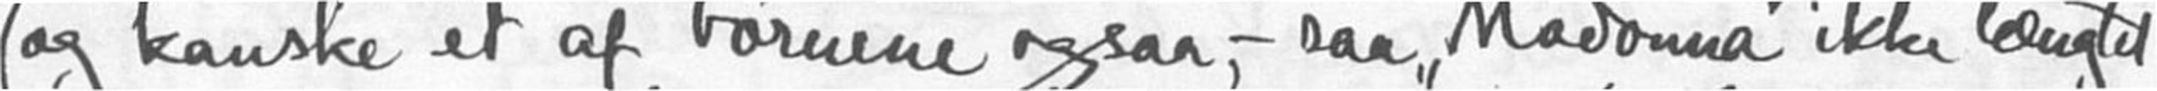(og kanske et af børnene ogsaa, - saa "Madonna" ikke længet
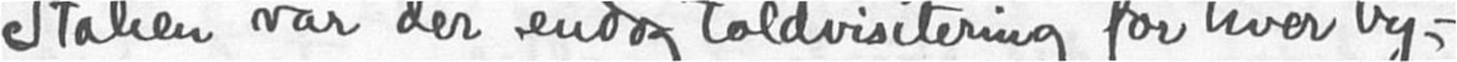Italien var der endog toldvisitering for hver by,-
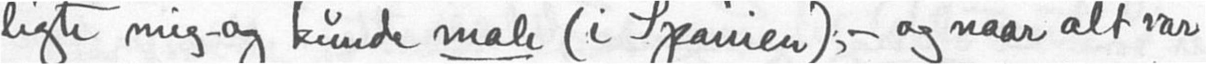ligte mig - og kunde male (i Spanien); -og naar alt var
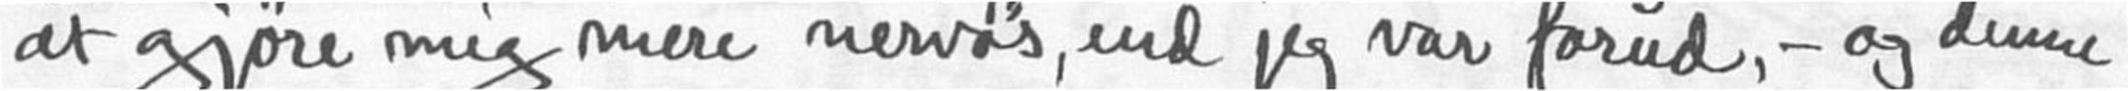at gjøre mig mere nervøs, end jeg var forud, - og denne
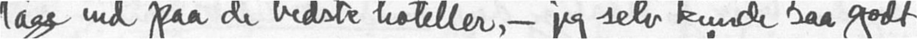tagte ind paa de bedste hoteller, - jeg selv kunde saa godt
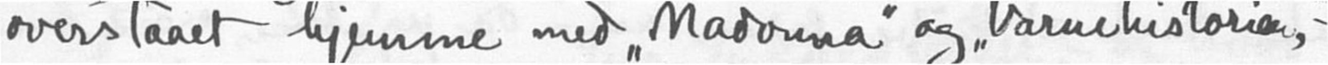overstaaet hjemme med "Madonna" og "barnehistorien", -
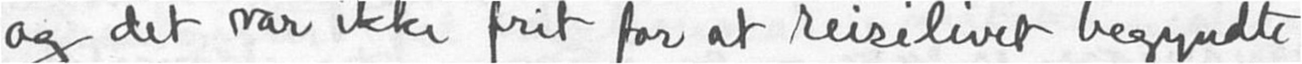og det var ikke frit for at reiselivet begyndte
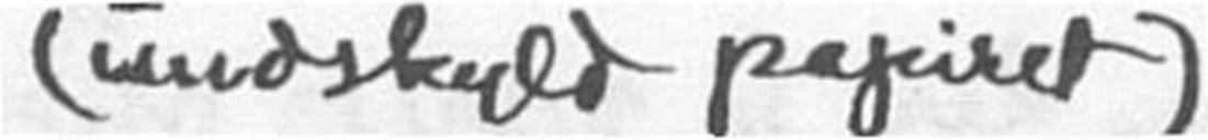(undskyld papiret)
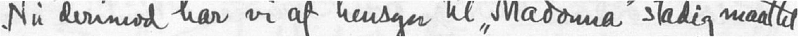Nu derimod har vi af hensyn til "Madonna" stadig maattet
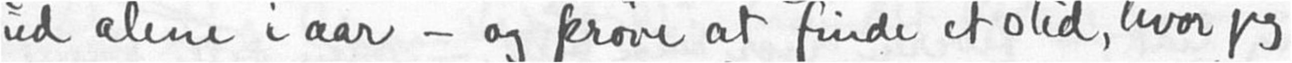ud alene i aar - og prøve at finde et sted, hvor jeg
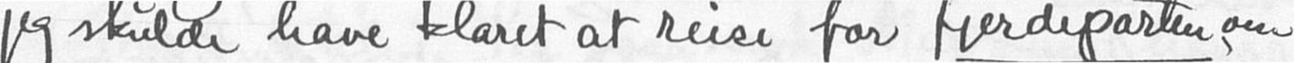jeg skulde have klaret at reise for fjerdeparten om
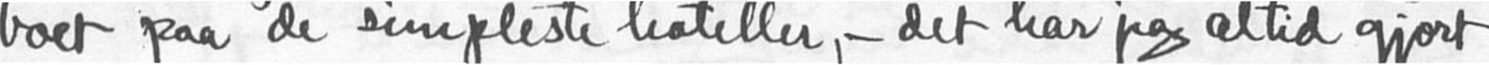boet paa de simpleste hoteller, - det har jeg altid gjort
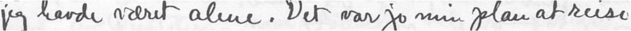jeg havde været alene. Det var jo min plan at reise
